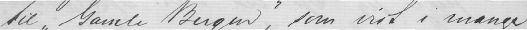til "Gamle Bergen", som vist i mange
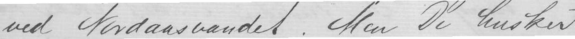ved Nordaasvandet. Men De husker
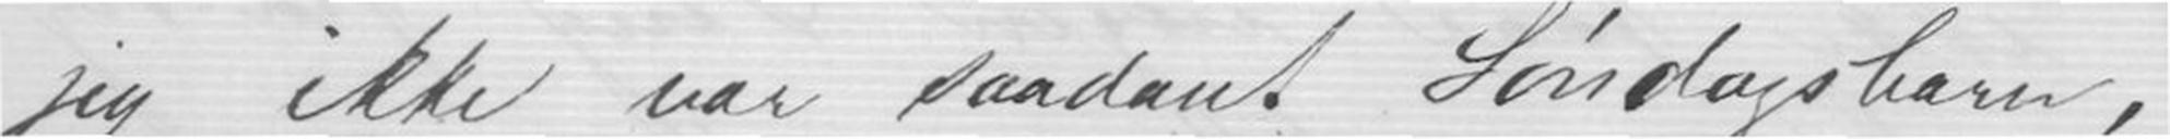jeg ikke var saadant Søndagsbarn,
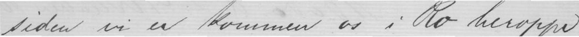siden vi er kommen os i Ro heroppe
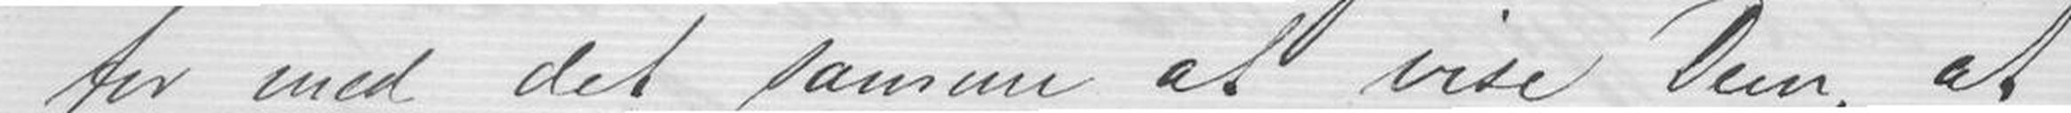for med det samme at vise Dem, at
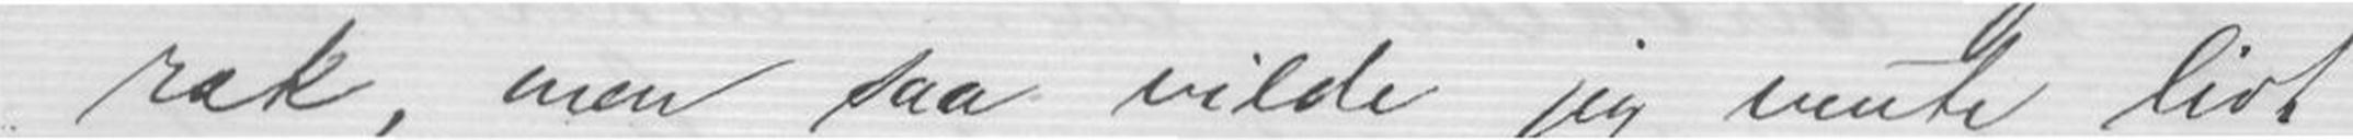rak, men saa vilde jeg vente lidt
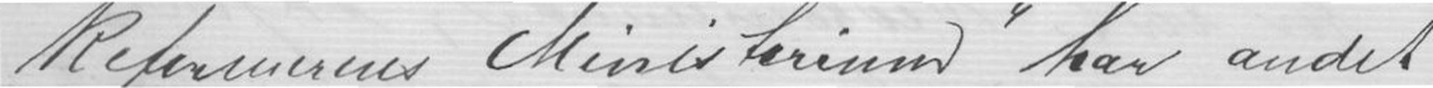"Reformerens Ministerium" har andet
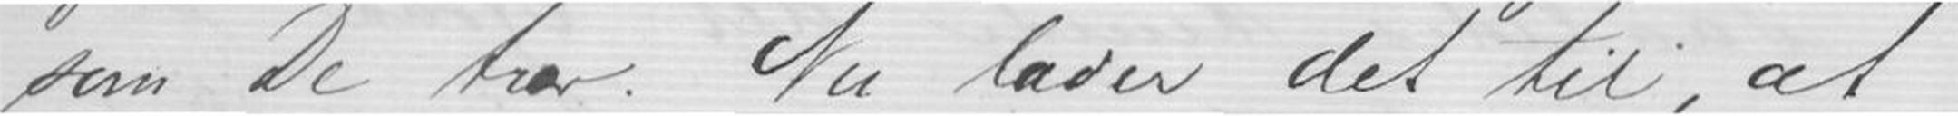som De tror. Nu lader det til, at
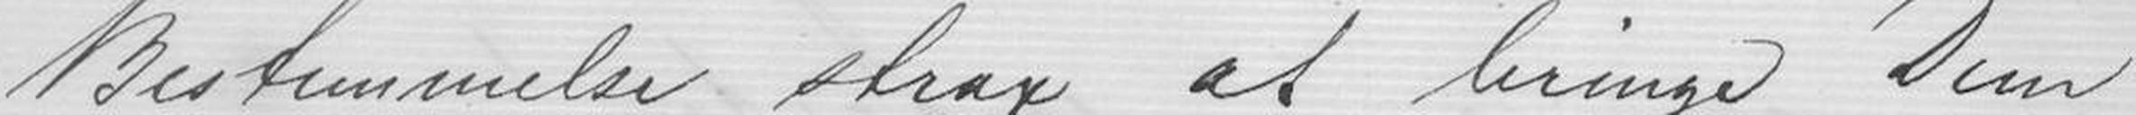Bestemmelse strax at bringe Dem
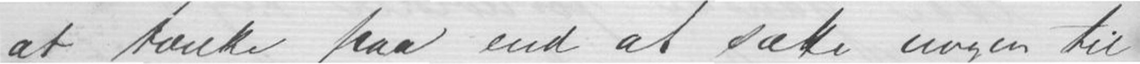at tænke paa end at sætte nogen til
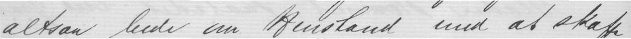altsaa bede om Henstand med at skaffe
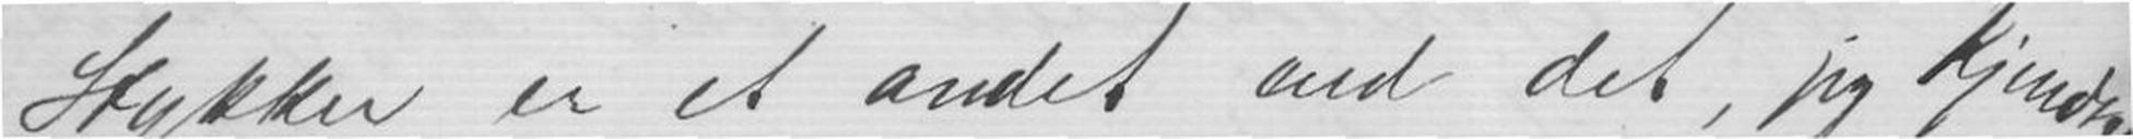Stykker er et andet end det, jeg kjender
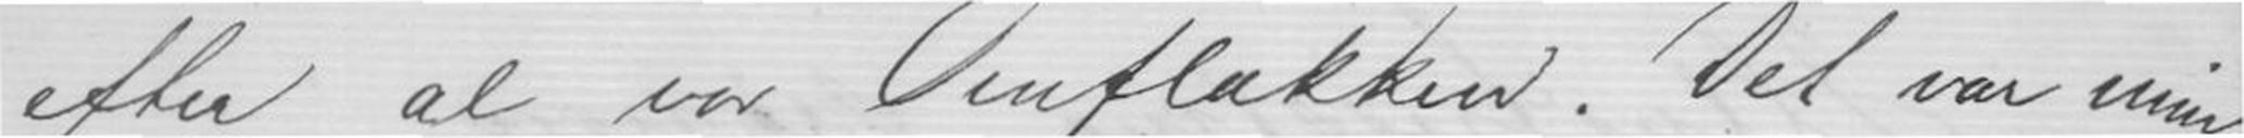efter al vor Omflakken. Det var min
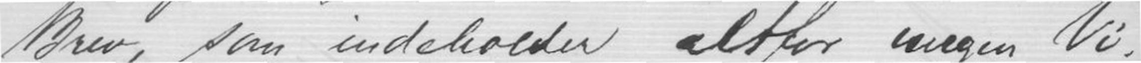Brev, som indeholder altfor megen Vi-
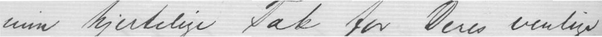min hjertelige Tak for Deres venlige
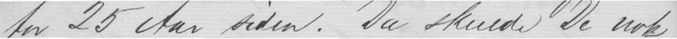for 25 Aar siden. Da skulde De nok
